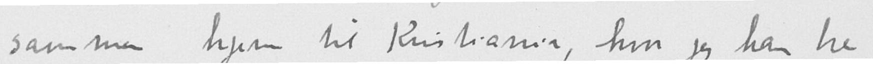sammen hjem til Kristiania, hvor jeg kan ha
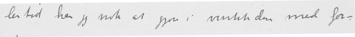lertid har jeg nok at gjøre i ventetiden med for-
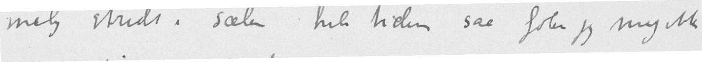melig stridt i sælen hele tiden saa føler jeg mig ikke
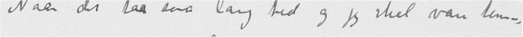Naar det tar saa lang tid og jeg skal være tem-
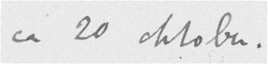ca 20 oktober.
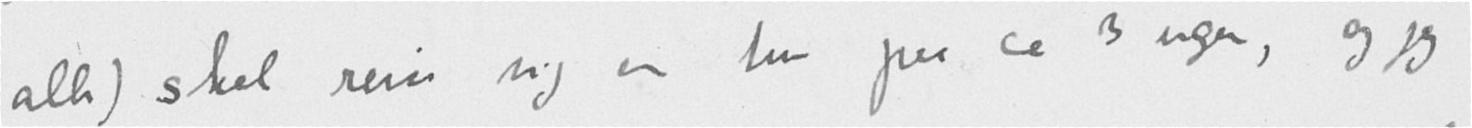alle) skal reise sig en tur paa ca 3 uger, og jeg
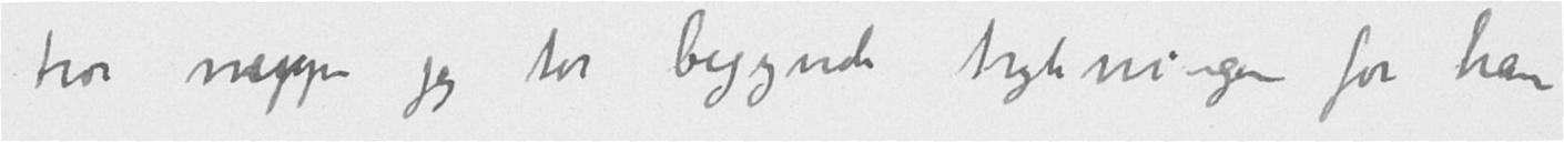tror næppe jeg tør begynde trykningen før han
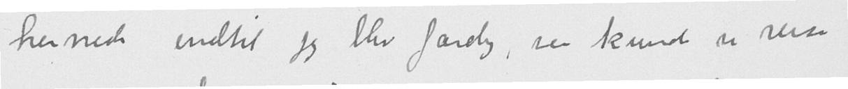hernede indtil jeg blev færdig, saa kunde vi reise
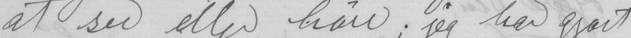at see eller høre; jeg har gjort
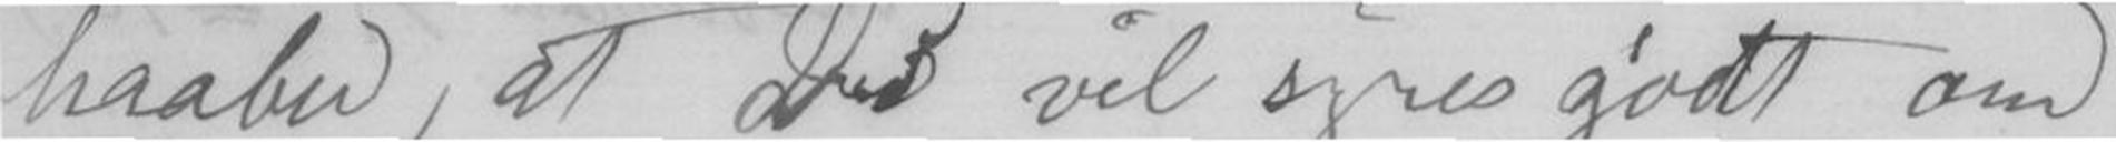haaber, at Du vil synes godt om
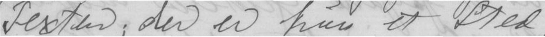Texten; der er kun et Sted,
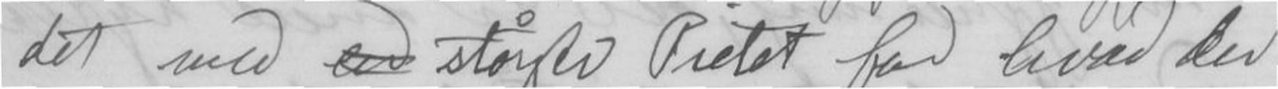det med en største Pietet for hvad der
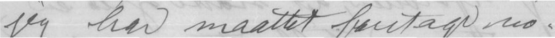jeg har maattet foretage no-
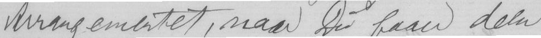Arrangementet, naar Du faar dem
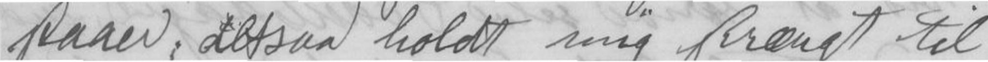staaer; altsaa holdt mig strængt til
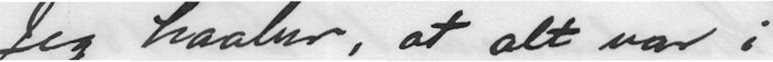Jeg haaber, at alt var i
